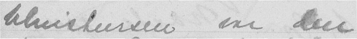Christensen var den
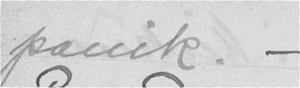panik. -
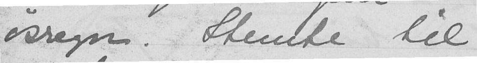øsregn. Stemte til
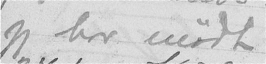jeg har mødt.
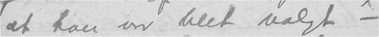at han var blit valgt -
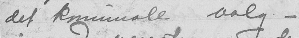det komunale valg. -
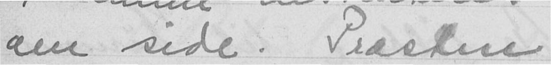om side. Præsten
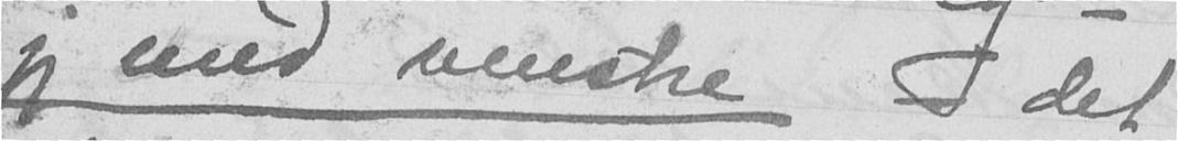jeg med venstre - det
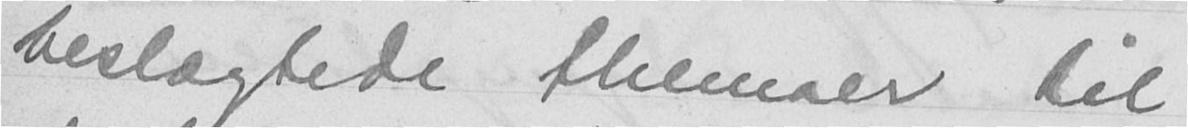beslægtede themaer til
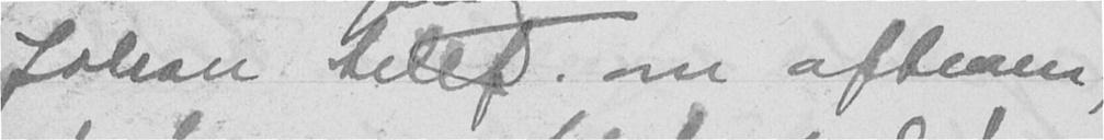Johan telef. om aftenen,
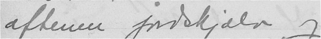aftenen jordskjælv og
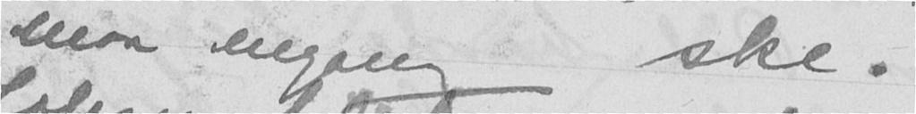maa engang ske.
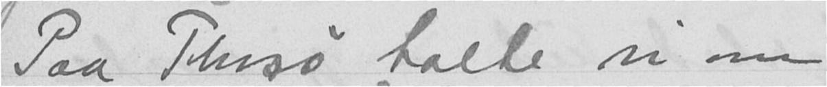Paa Thorsø talte vi om
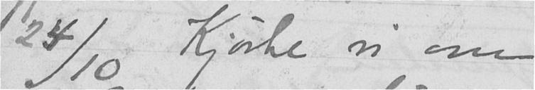24/10 Kjørte vi om
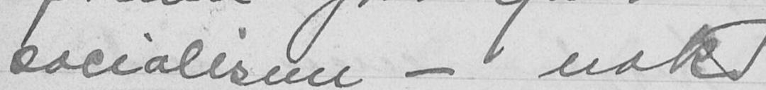socialisme - nok
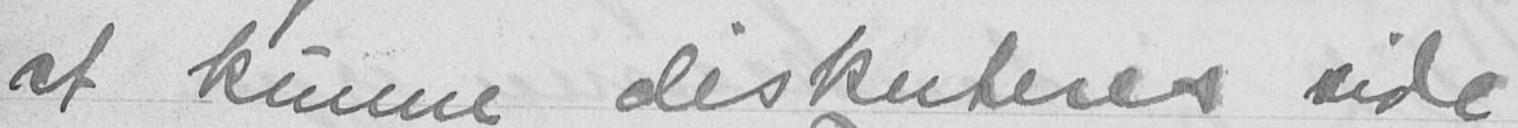at kunne diskuteres side
